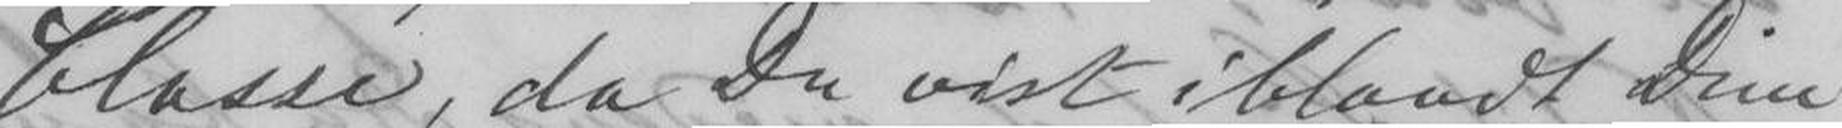Classe, da Du vist iblandt Dine
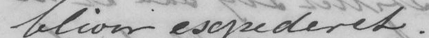forlader bliver expederet
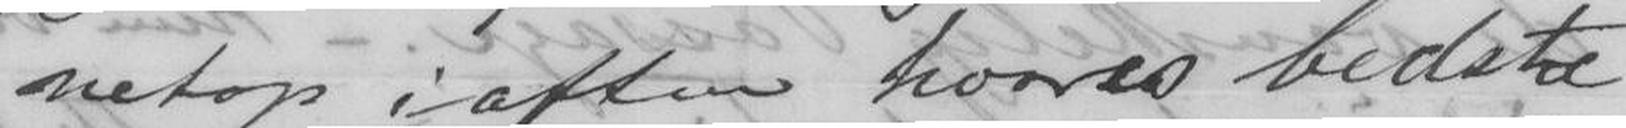netop iaften hvores bedste
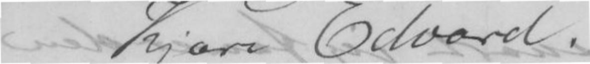Kjære Edvard.
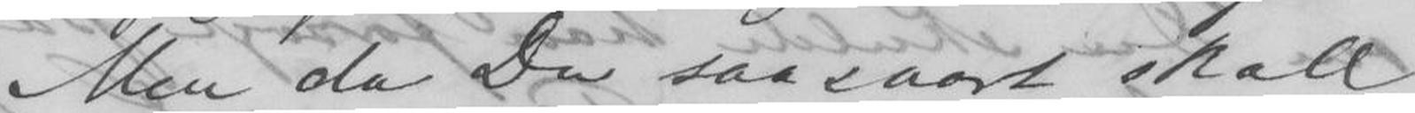Men da Du saasnart skall
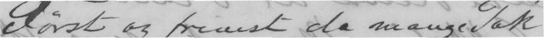Først og fremst da mange Tak
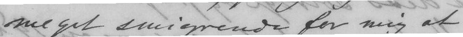meget smigrende for mig at
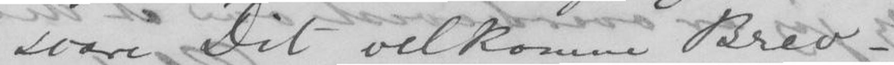svare Dit velkomne Brev
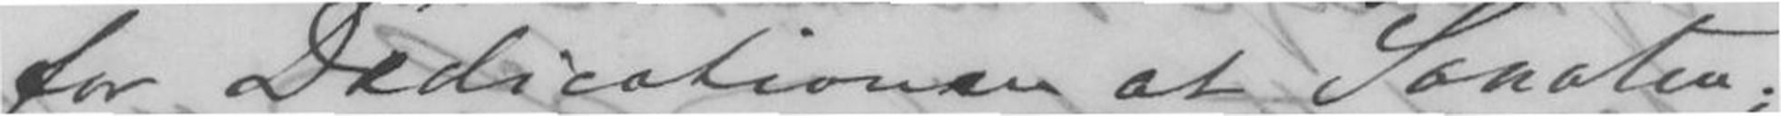for Dedicationen at Sonaten;
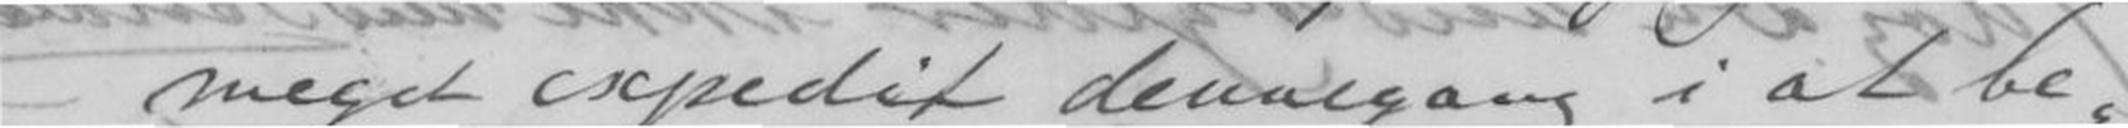meget expedit dennegang i at be-
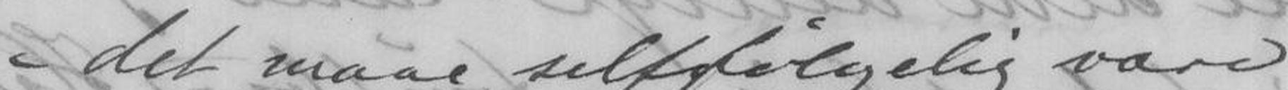det maae selvfølgelig være
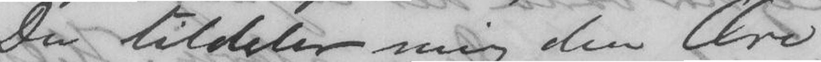Du tildeled mig den Ære
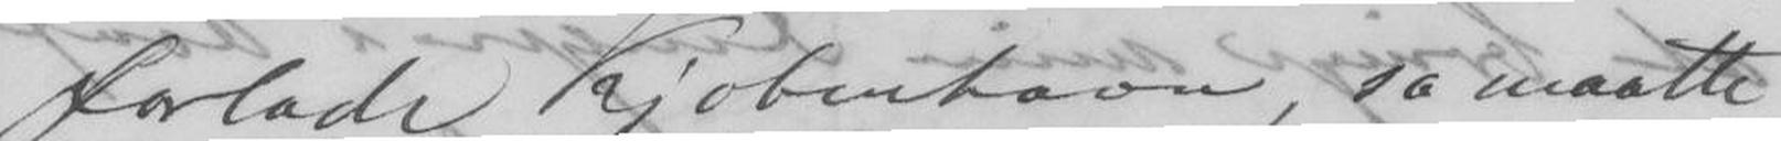forlade Kjøbenhavn, so maatte
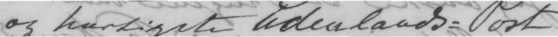og hurtigste Udenlands-Post
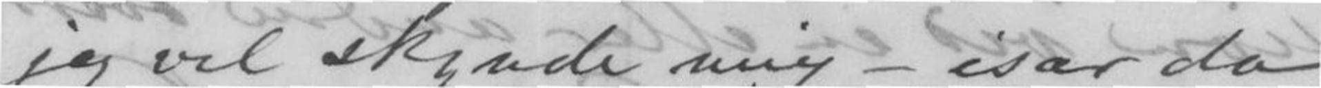jeg vel skynde mig - især da
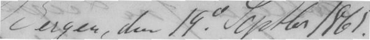Bergen, den 19d Septbr 1865.
